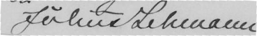Julius Lehmann
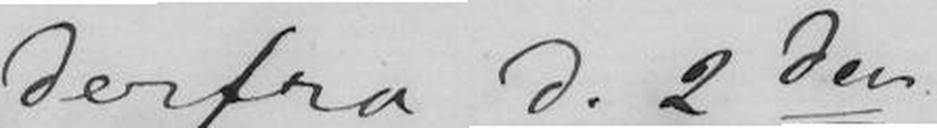derfra d. 2den.
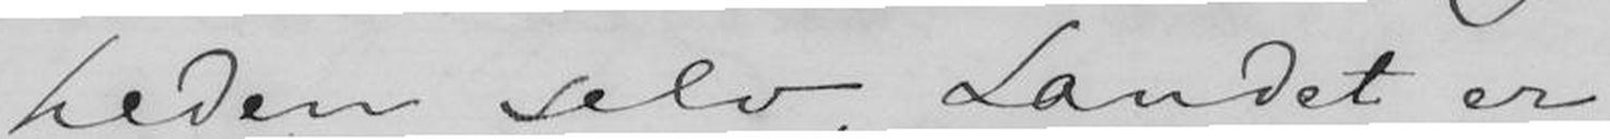heden selv, Landet er
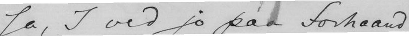Ja, I ved jo paa forhaand,
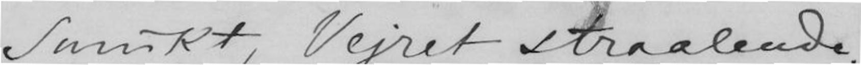Smukt, Vejret straalende.
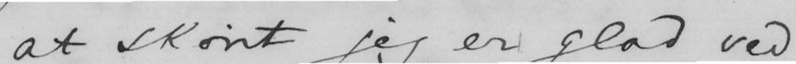at skønt jeg er glad ved
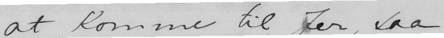at komme til Jer, saa
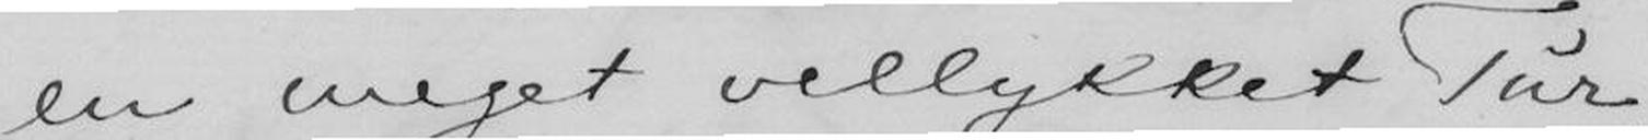en meget vellykket Tur.
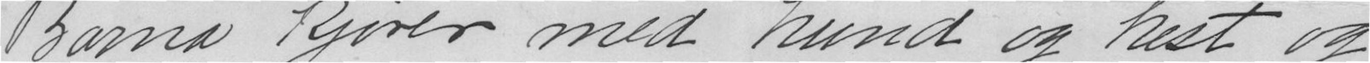Barna kjører med hund og hest og
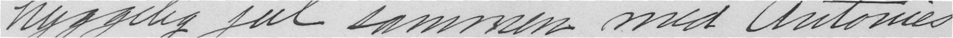hyggelig jul sammen med Antonies
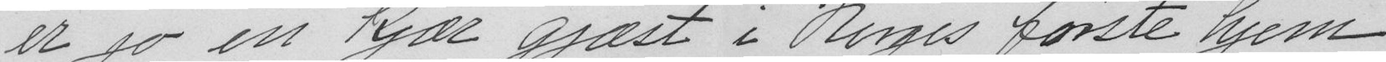er jo en kjær gjæst i Norges første hjem
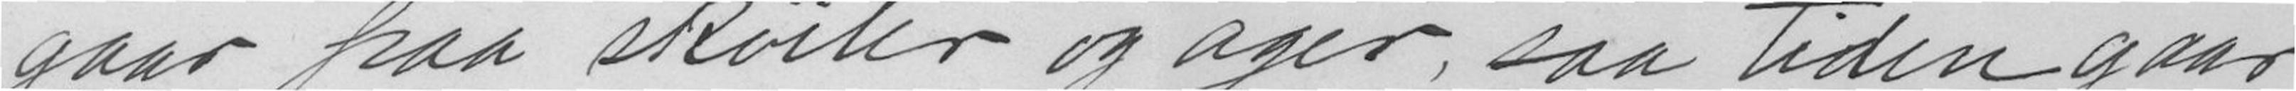gaar paa skøiter og ager, saa tiden gaar
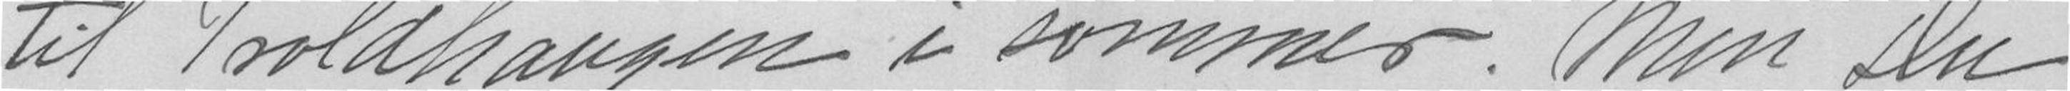til Troldhaugen i sommer. Men Du
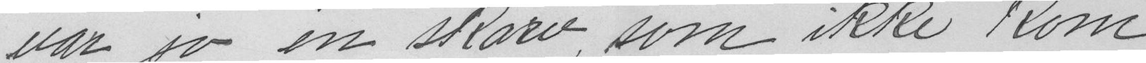var jo en skarv, som ikke kom
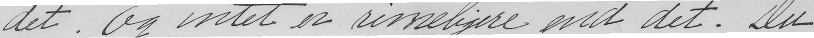det. Og intet er rimeligere end det. Du
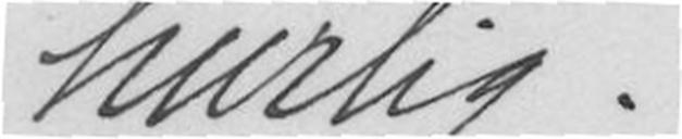hurtig.
